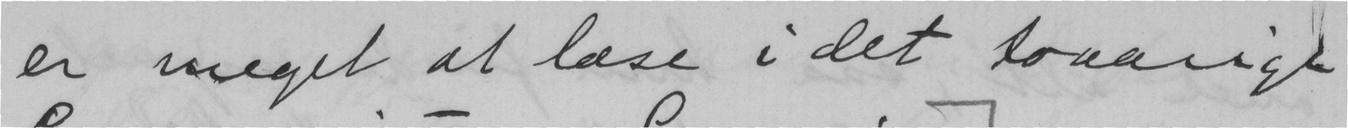er meget at læse i det toaarige
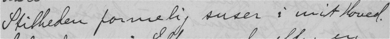Stilheden formelig suser i mit Hoved.
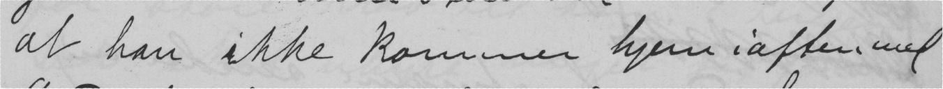at han ikke kommer hjem i aften med
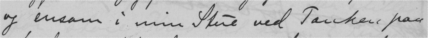og ensom i min Stue ved Tanken paa
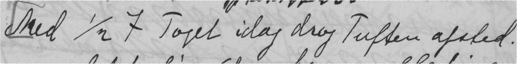Med ½ 7 Toget idag drog Tuften afsted.
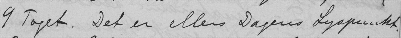9 Toget. Det er ellers Dagens Lyspunkt.
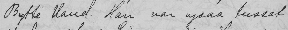Bytte Vand. Han var ogsaa tusset
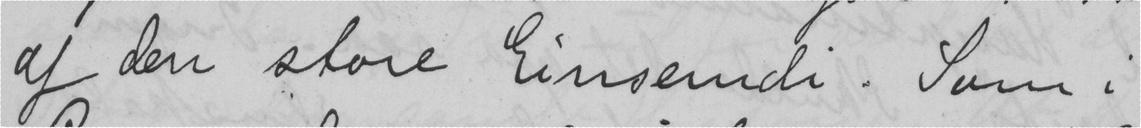af den store Einsemdi. Som i
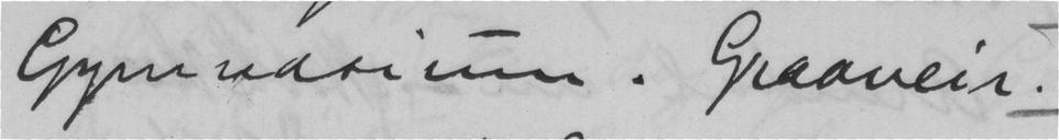Gymnasium. Graaveir.
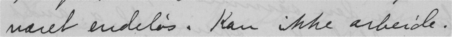været endeløs. Kan ikke arbeide.
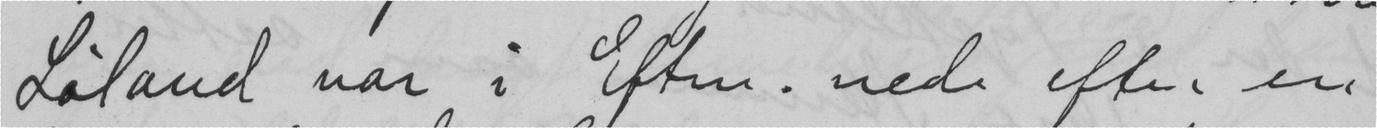Løland var i efterm. nede efter en
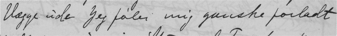Vægge ude Jeg føler mig ganske forladt
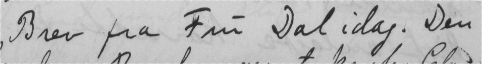Brev fra Fru Dal idag. Den
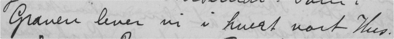Graven lever vi i hvert vort Hus.
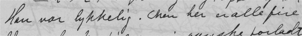Han var lykkelig. Men her er alle fire
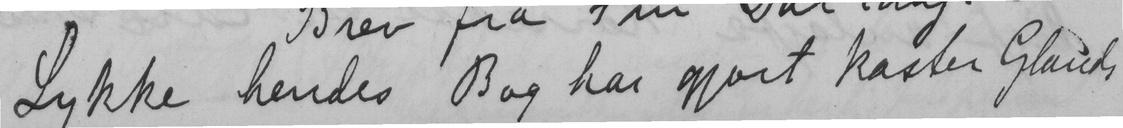Lykke hendes Bog har gjort kaster Glands
